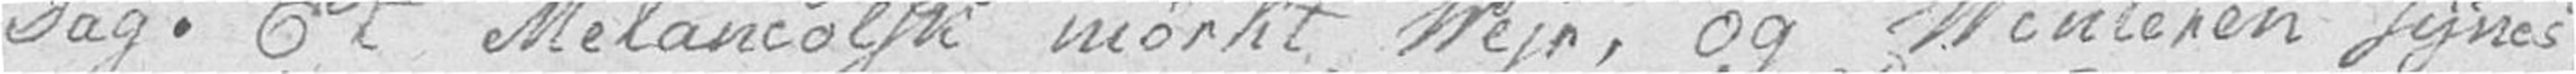Dag. Et Melancolsk mørkt Vejr, og Vinteren synes
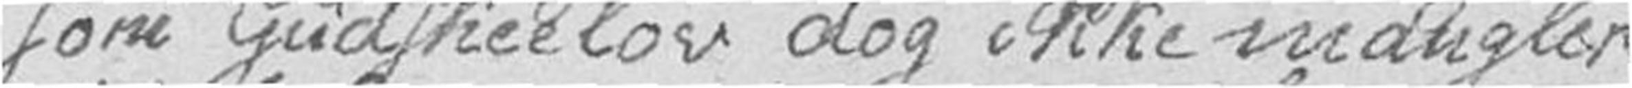som Gudskeelov dog ikke mangler
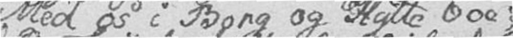Med os i Borg og Hytte boe:
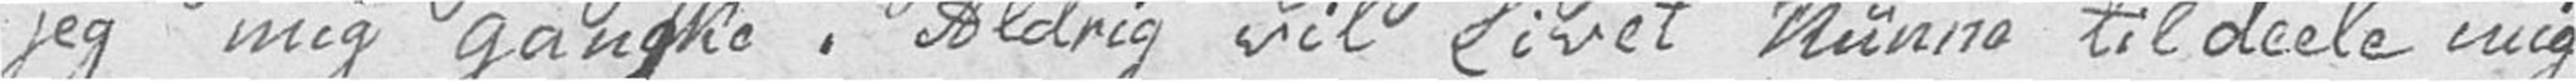jeg mig ganske. Aldrig vil Livet kunne tildeele mig
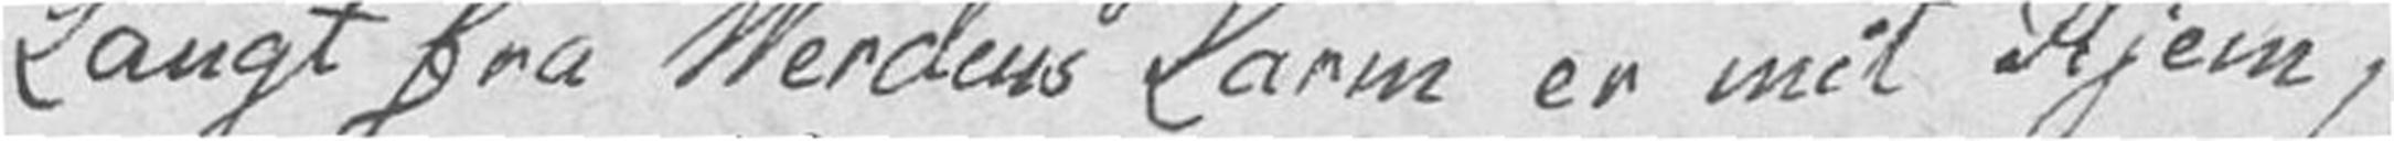Langt fra Verdens Larm er mit Hjem,
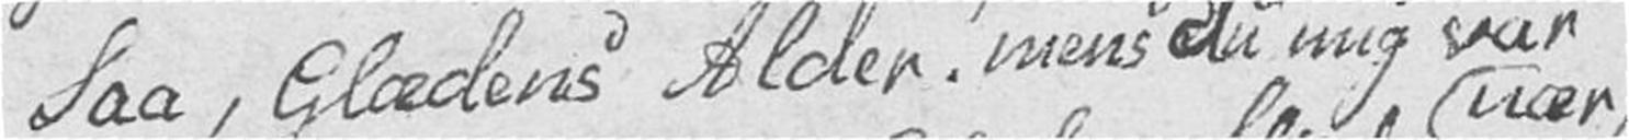Saa, Glædens Alder! mens du mig var nær,
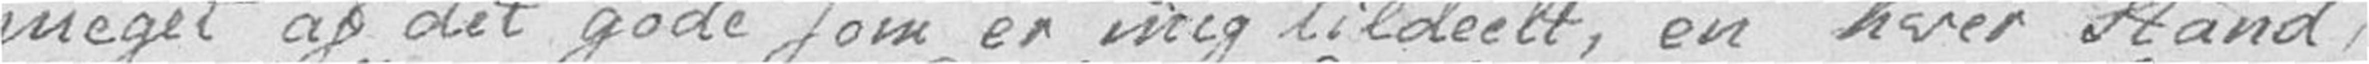meget af det gode som er mig tildeelt, en hver Stand,
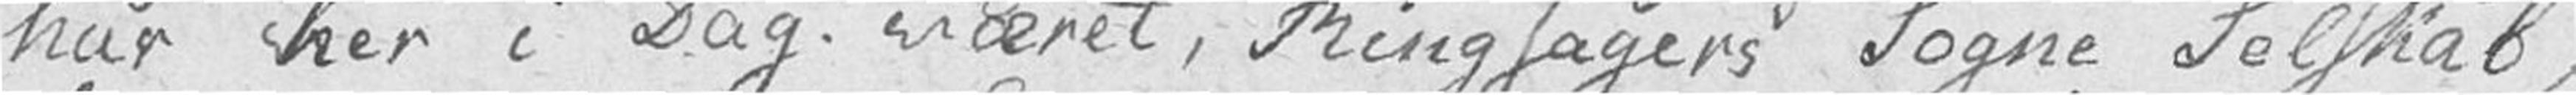har her i Dag været, Ringsagers Sogne Selskab,
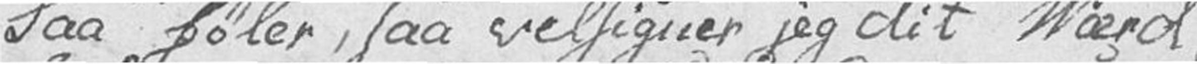Saa føler, saa velsigner jeg dit Værd
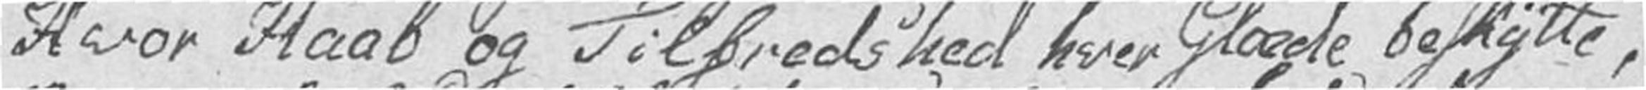Hvor Haab og Tilfredshed hver Glæde beskytte,
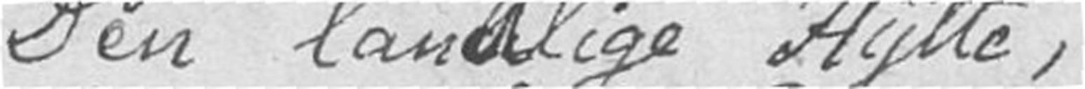Den landlige Hytte,
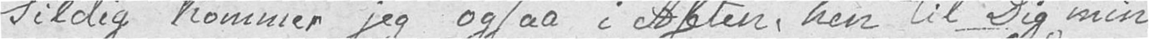Sildig kommer jeg ogsaa i Aften, hen til Dig min
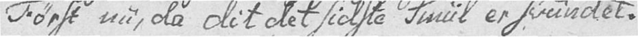Først nu, da dit det sidste Smiil er svundet.
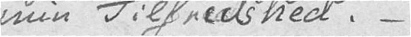min Tilfredshed. –
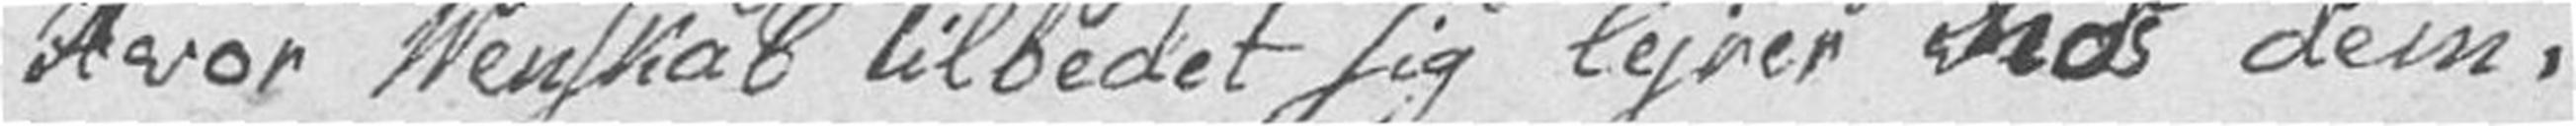Hvor Venskab tilbedet sig lejrer hos dem.
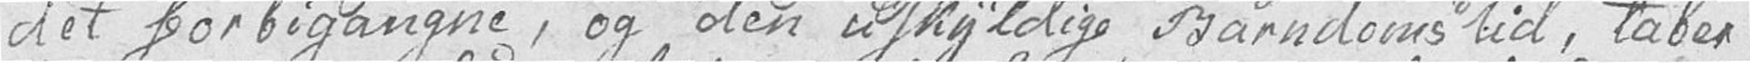det forbigangne, og den uskyldige Barndoms tid, taber
